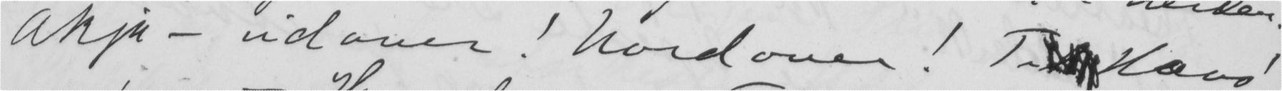Akja - udover! Nordover! Til Værs!
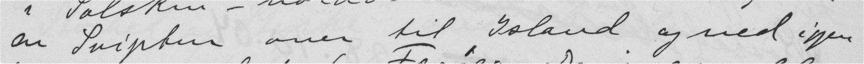en Sviptur over til Island og ned igen
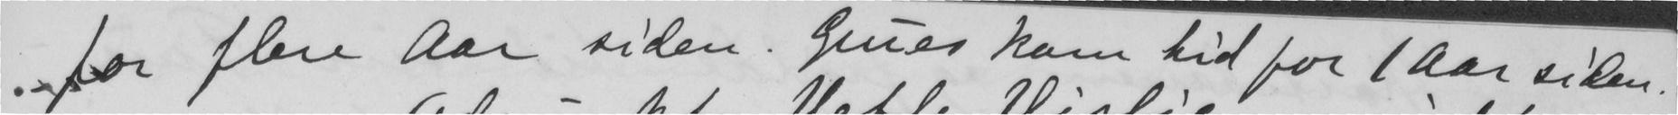for flere Aar siden. Grues kom hid for 1Aar siden.
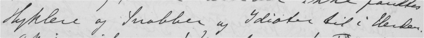Hyklere og Snobber og Idioter til i Verden.
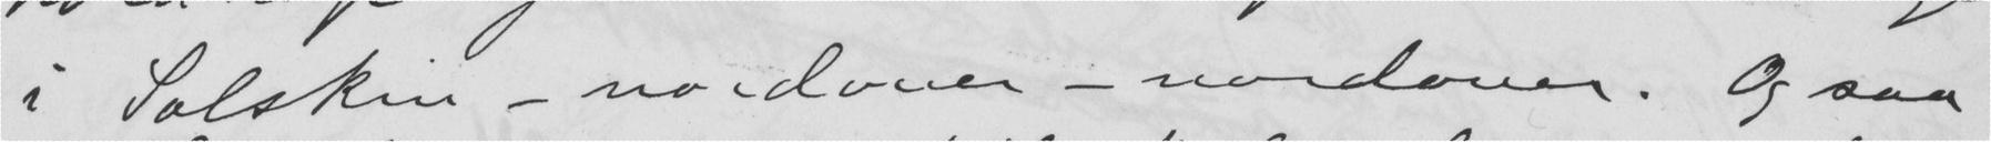i Solskin - nordover - nordover. Og saa
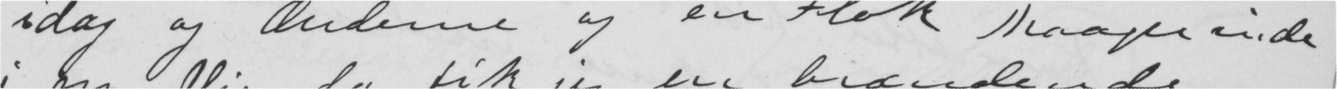idag og Ænderne og en Flok Maager ude
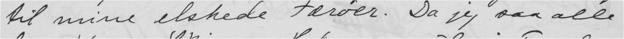til mine elskede Færøer. Da jeg saa alle
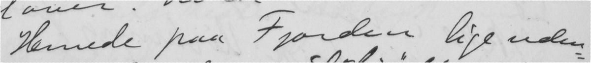Hernede paa Fjorden lige uden-
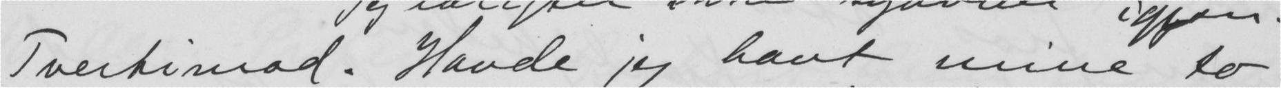Tvertimod. Havde jeg havt mine to
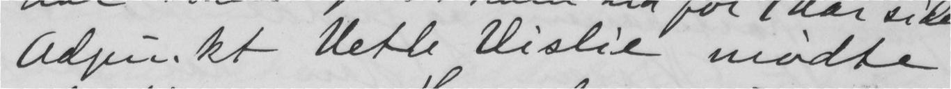Adjunkt Vetle Vislie mødte
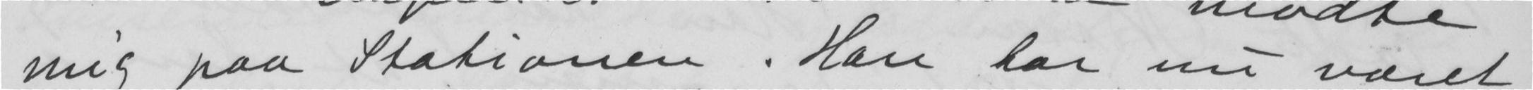mig paa Stationen. Han har nu været
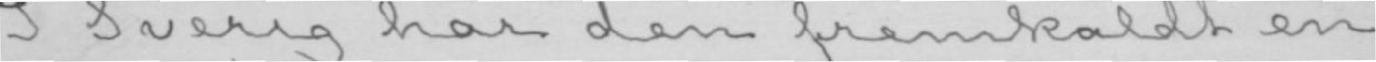I Sverig har den fremkaldt en
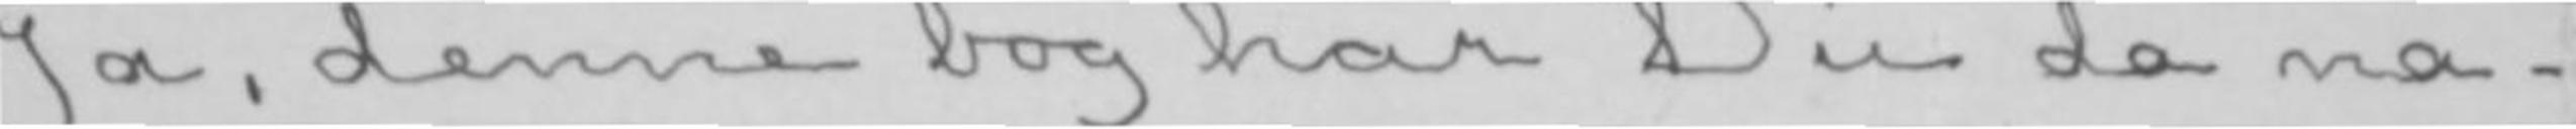Ja, denne bog har Du da na-
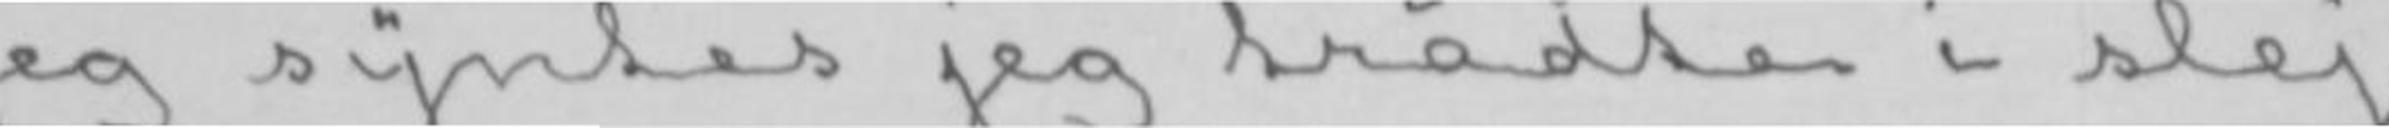jeg syntes jeg trådte i slejp
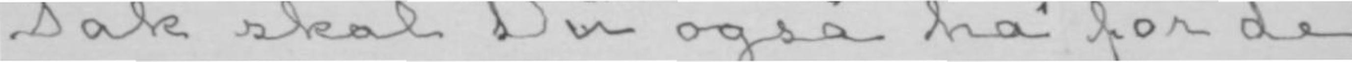Tak skal Du også ha&rapo; for de
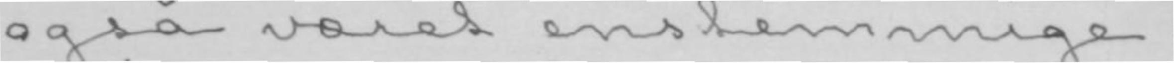også været enstemmige.
